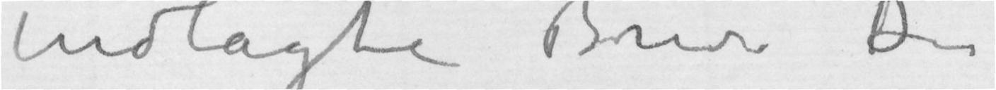indlagte Brev Du
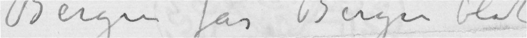Bergen får Bergen blot
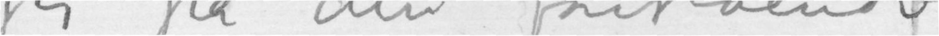jeg på den forestående
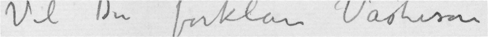Vil Du forklare Vasteson
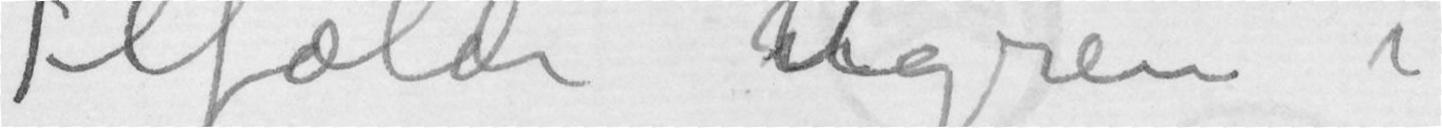Tilfælde Ugreie i
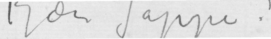Kjære Jappe!
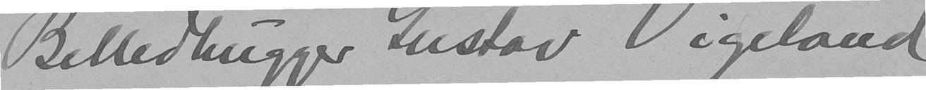Billedhugger Gustav Vigeland
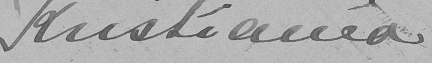Kristiania
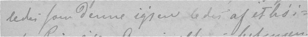ledes som denne igjen ledes af et høi-
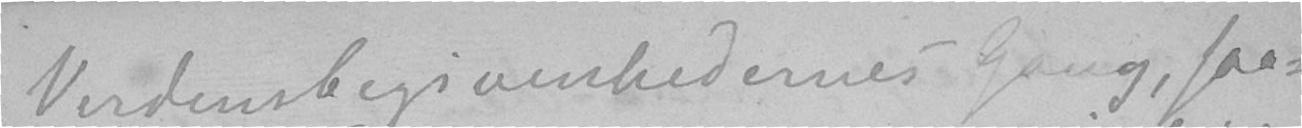Verdensbegivenhedernes Gang, saa-
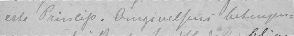este Princip. Omgivelsens betingen-
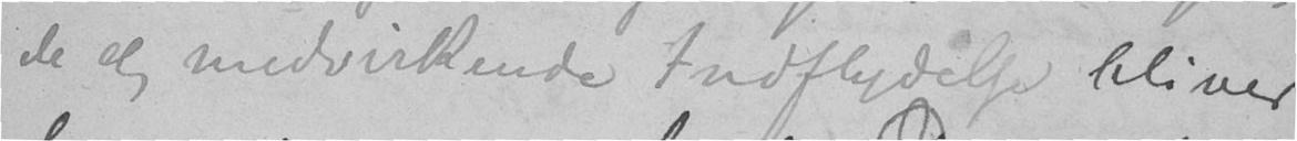de og medvirkende Indflydelse bliver
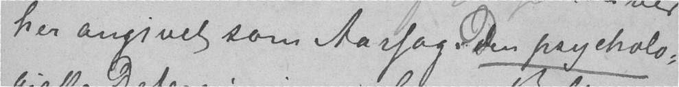her angivet som Aarsag. Den psycholo-
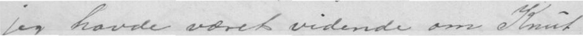jeg havde været vidende om Knut
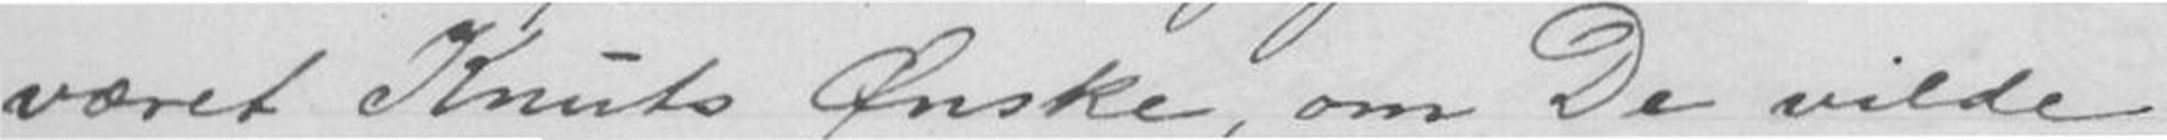været Knuts Ønske, om De vilde
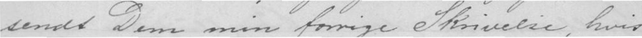sendt Dem min forrige Skrivelse, hvis
